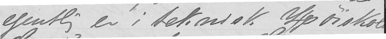egentlig er i teknisk Høiskole.
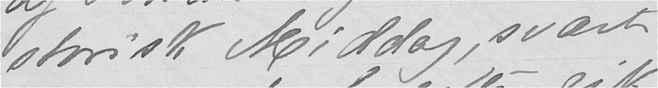storisk Middag, svært
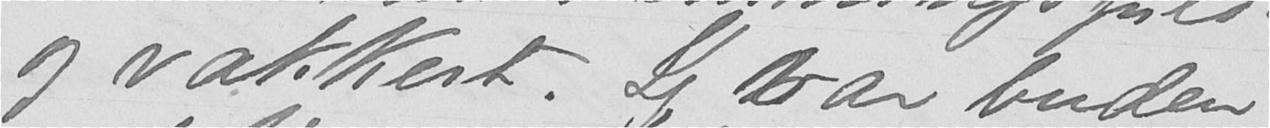og vakkert. Jeg var buden
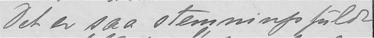Det er saa stemningsfuldt
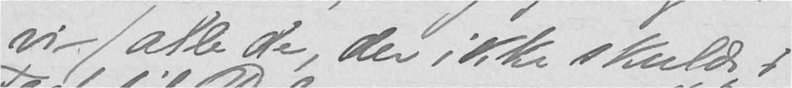vi - (alle de, der ikke skulde i
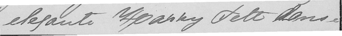elegante Harry Fett danse.
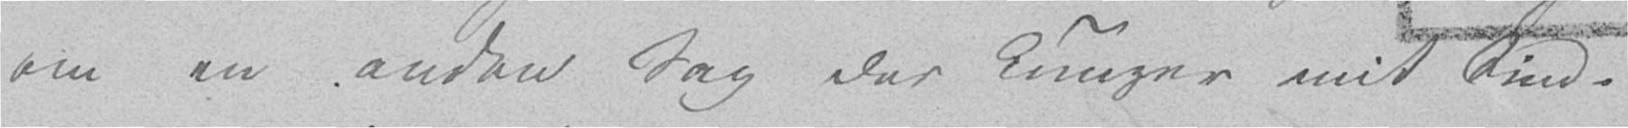om en anden Sag der knuger mit Sind.
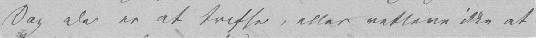Dag De er at treffe, eller rettere ikke at
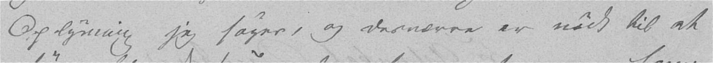Oplysning jeg søger, og desværre er nødt til at
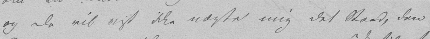og De vil vist ikke nægte mig det Raad, den
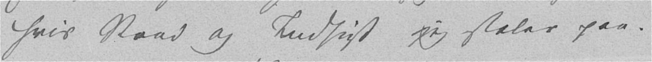hvis Raad og Indsigt jeg stoler paa.
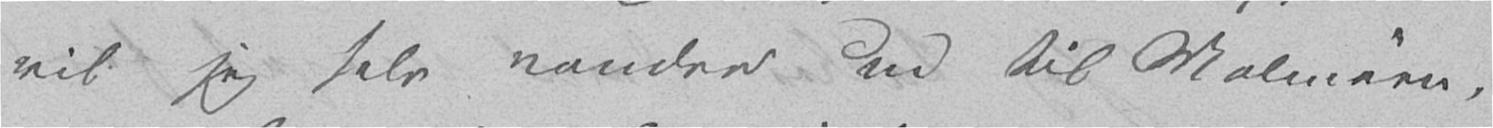vil jeg selv vandre ud til Malmøen,
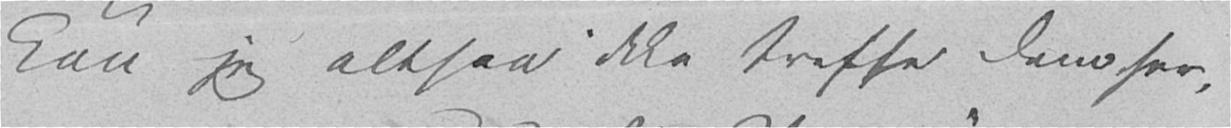Kan jeg altsaa ikke treffe Dem her,
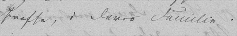treffe, i Deres Familie.
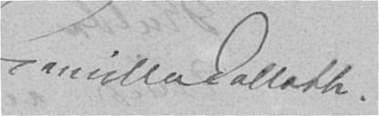Camilla Collett.
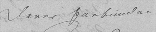Deres forbundne
